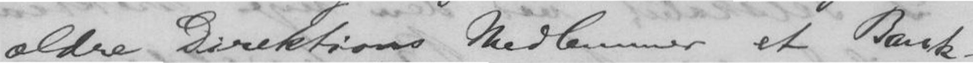ældre Direktions Medlemmer et Bank-
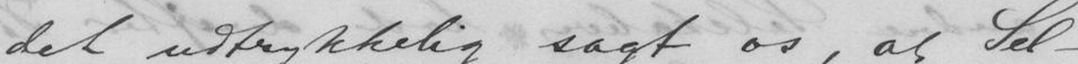det udtrykkelig sagt os, at Sel-
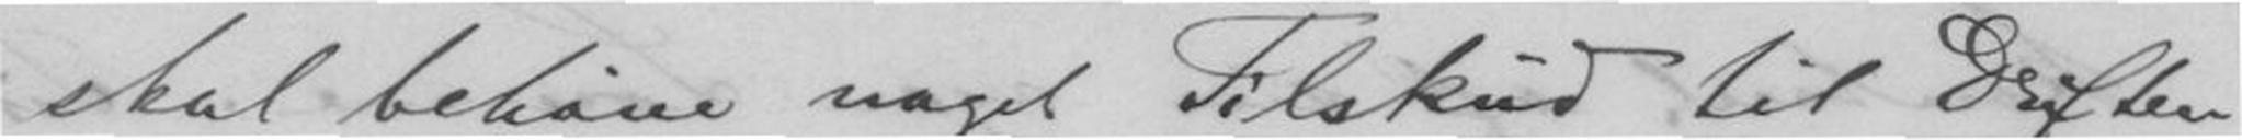skal behøve noget Tilskud til Driften
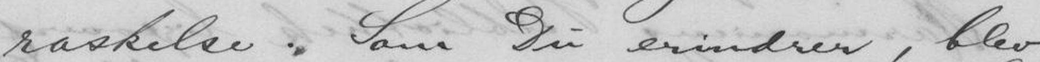raskelse. Som Du erindrer, blev
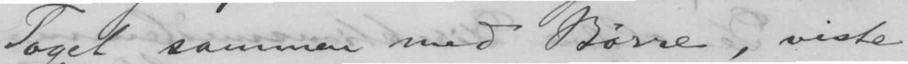Toget sammen med Børre, viste
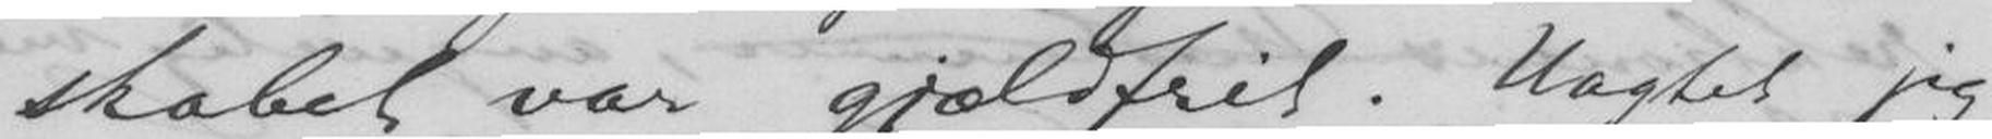skabet var gjældfrit. Uagtet jeg
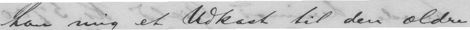han mig et Udkast til den ældre
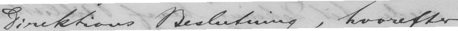Direktions Beslutning, hvorefter
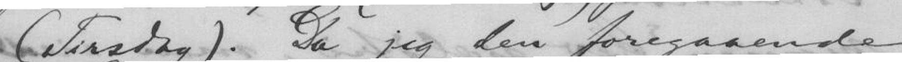(Tirsdag). Da jeg den foregaaende
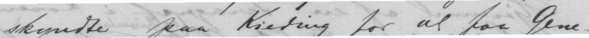skyndte paa Kieding for at faa Gene-
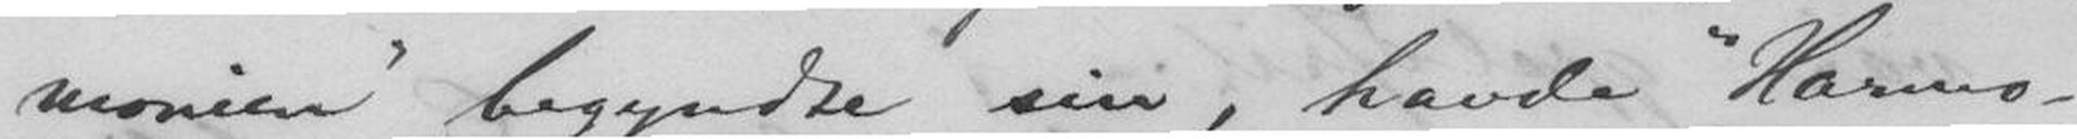monien" begyndte sin, havde "Harmo-
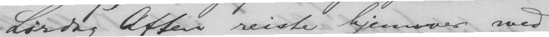Lørdag Aften reiste hjemover med
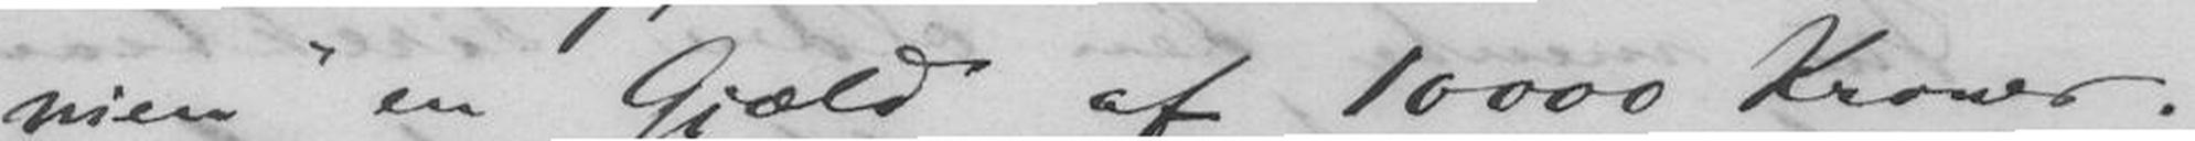nien" en Gjæld af 10000 Kroner.
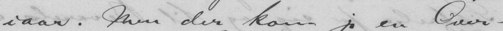iaar. Men der kom jo en Over-
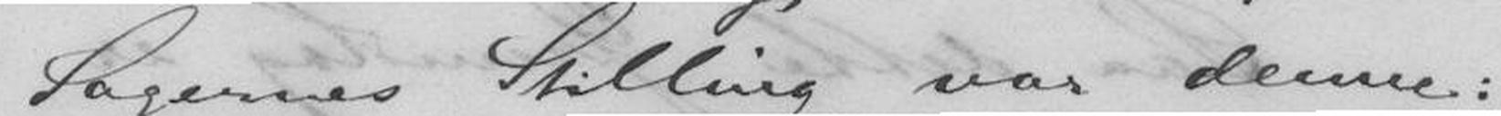denne Sagens Stilling var denne:
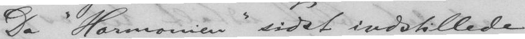Da "Harmonien" sidst indstillede
